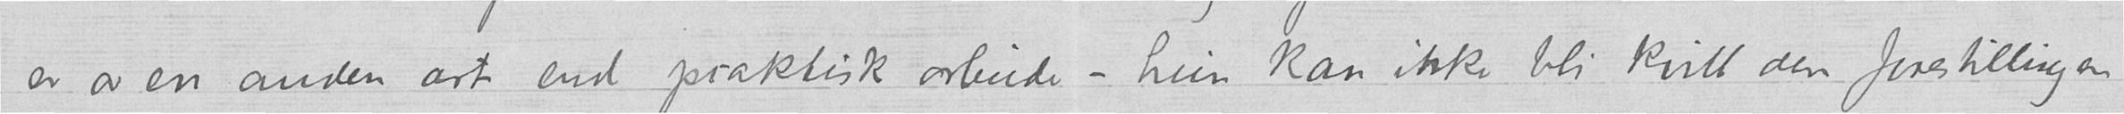er av en anden art end praktisk arbeide – hun kan ikke bli kvitt den forestillingen
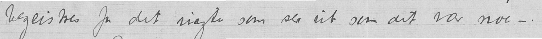begeistres for det uægte som ser ut som det var noe –.
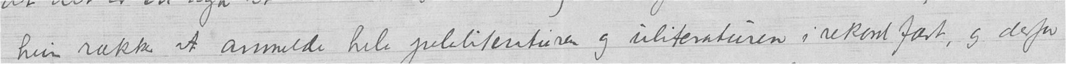hun rækker at anmelde hele juleliteraturen og uliteraturen i rekordfart, og derfor
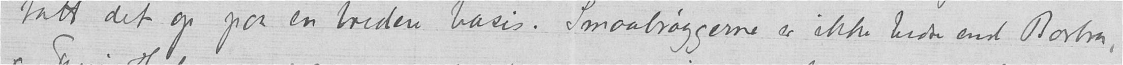tatt det op paa en bredere basis. Smaabrøggerne er ikke bedre end Barbra,
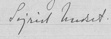Sigrid Undset.
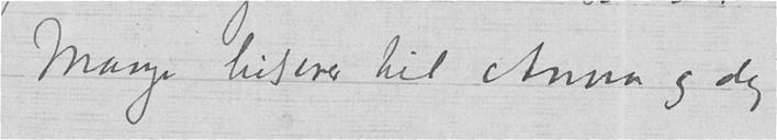Mange hilsener til Anna og dig
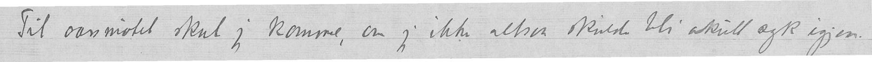Til aarsmøtet skal jeg komme, om jeg ikke altsaa skulde bli akutt syk igjen.
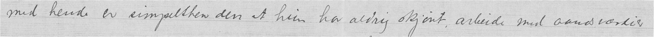med hende er simpelthen den at hun har aldrig skjønt, arbeide med aandsværdier
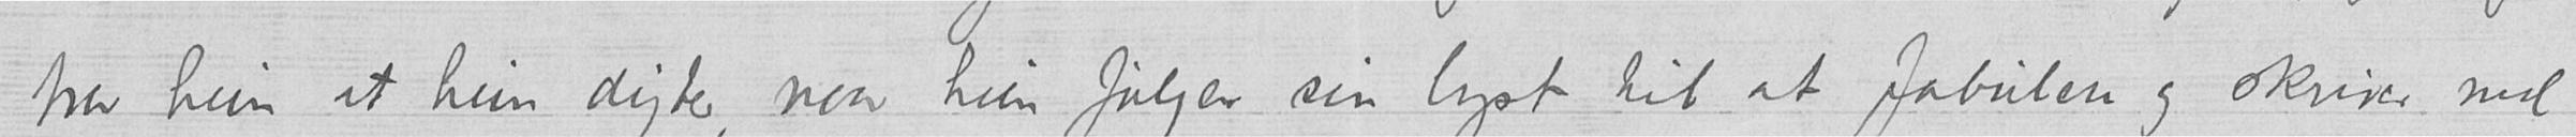tror hun at hun digter, naar hun følger sin lyst til at fabulere og skriver ned
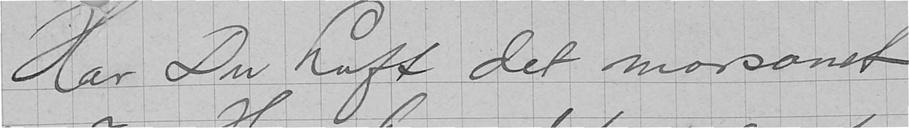Har Du haft det morsomt
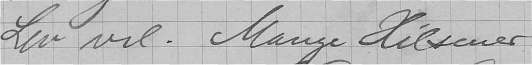Lev vel. Mange Hilsener
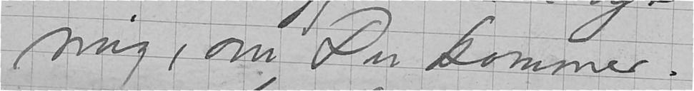mig, om Du kommer.
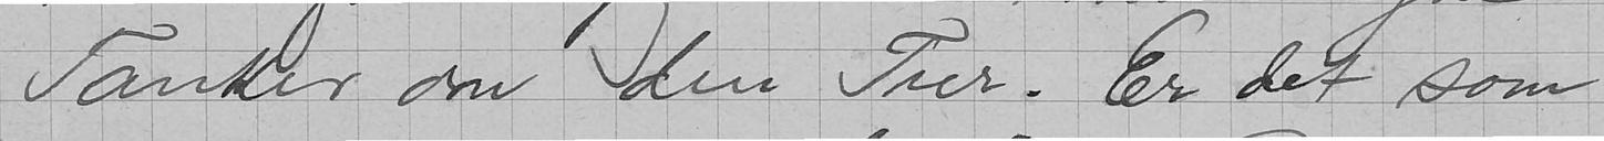Tanker om den Tur. Er det som
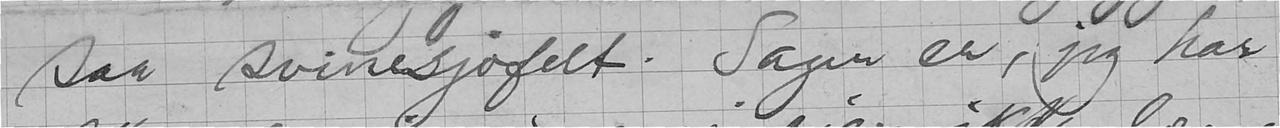saa svinesjofelt. Sagen er, jeg har
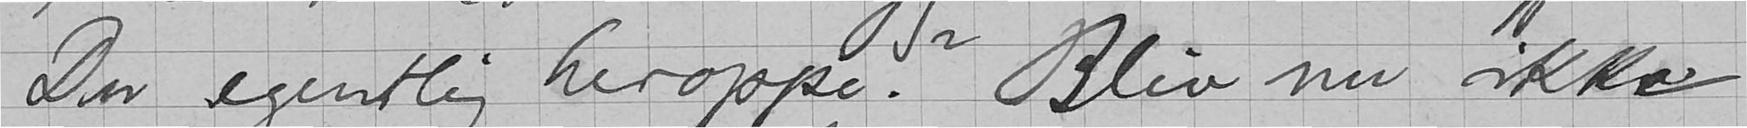Du egentlig heroppe? Bliv nu ikke
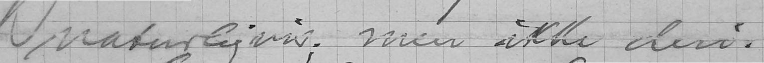naturligvis; men ikke deri.
